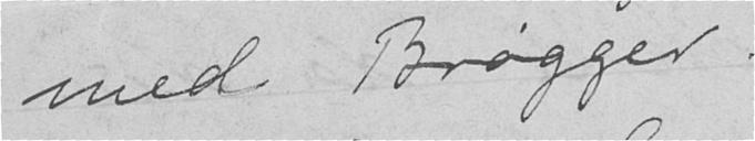med Brøgger.
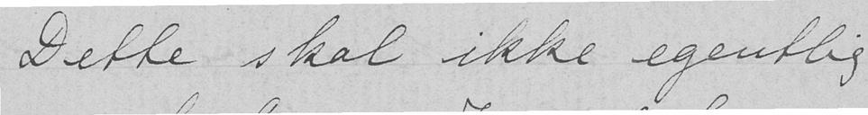Dette skal ikke egentlig
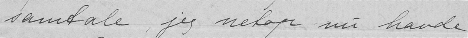samtale, jeg netop nu havde
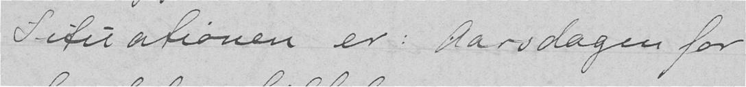Situationen er: aarsdagen for
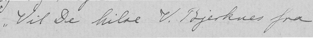"Vil De hilse V. Bjerknes fra
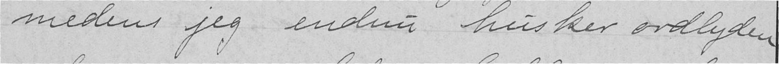medens jeg endnu husker ordlyden
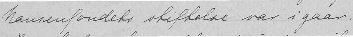Nansenfondets stiftelse var igaar.
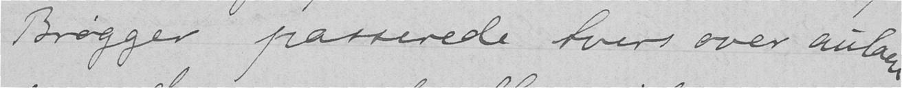Brøgger passerede tvers over aulaen
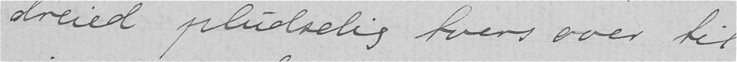dreied pludselig tvers over til
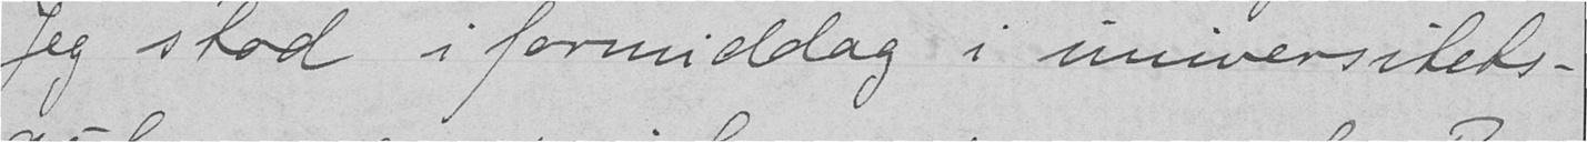Jeg stod i formiddag i universitets-
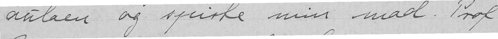aulaen og spiste min mad. Prof.
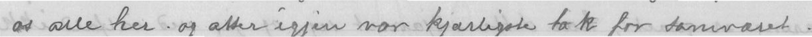os alle her og atter igjen vor kjærligste tak for samværet.
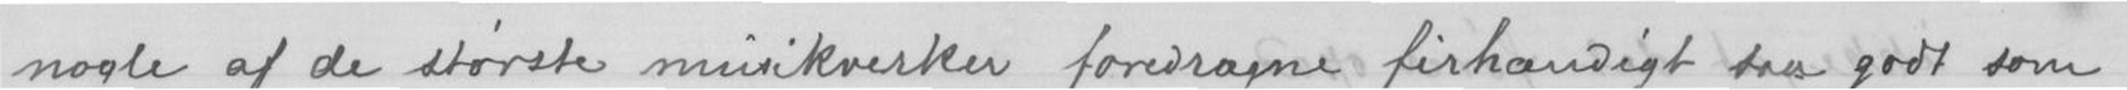nogle af de største musikverker foredragne firhændigt saa godt som
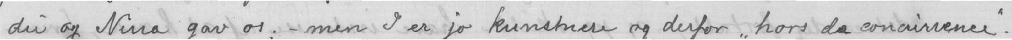det du og Nina gav os; - men I er jo kunstnere og derfor "trors de conairvence".
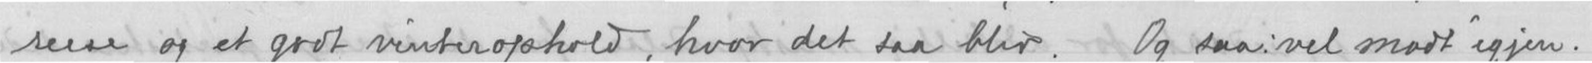reise og et godt vinterophold, hvor det saa blir. Og saa vel mødt igjen.
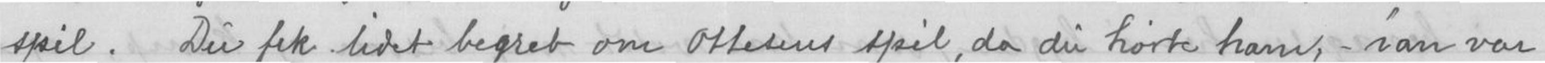spil. Du fik lidet begreb om Ottesens spil, da du hørte ham, - han var
